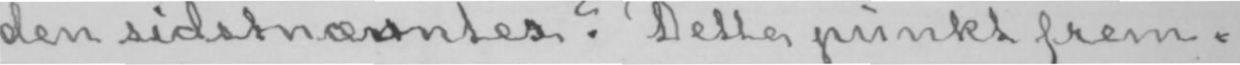den sidstnænvnters? Dette punkt frem-
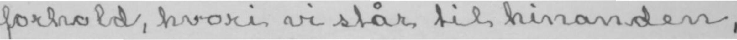forhold, hvori vi står til hinanden,
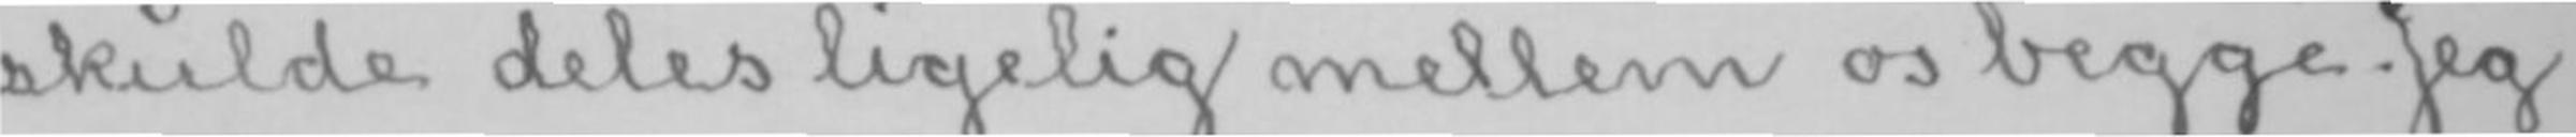skulde deles ligelig mellem os begge. Jeg
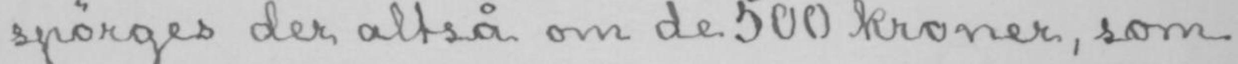spørges der altså om de 500 kroner, som
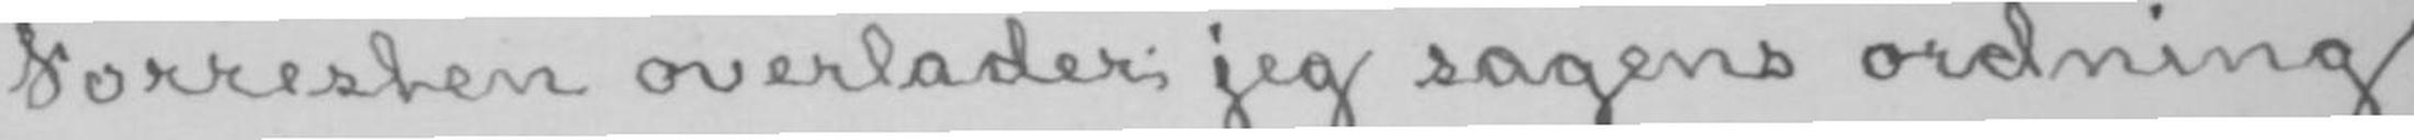Forresten overlader jeg sagens ordning
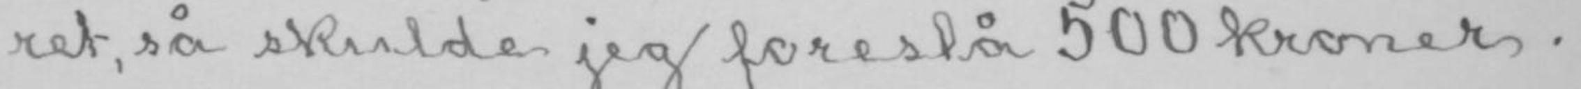ret, så skulde jeg foreslå 500 kroner.
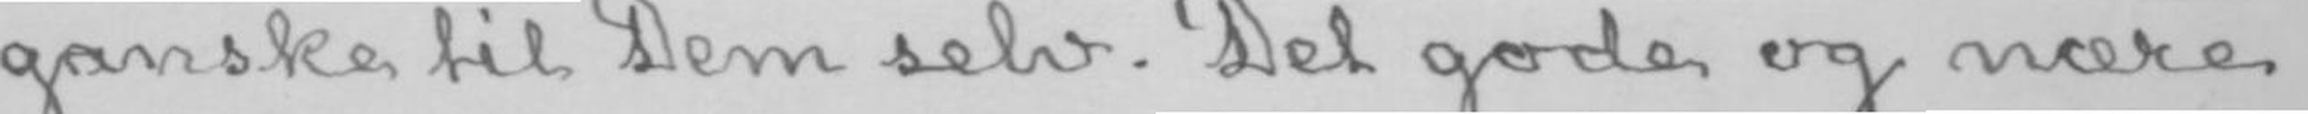ganske til Dem selv. Det gode og nære
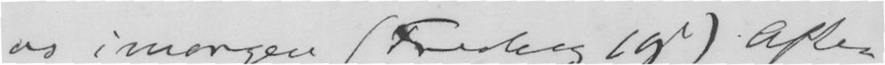os imorgen (Fredag 19de) Aften
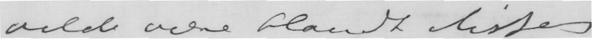vilde være blandt listens
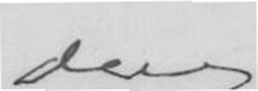deres
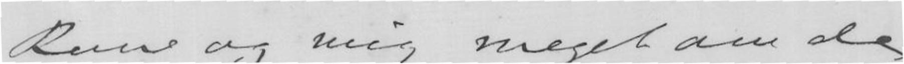Kone og mig meget om de
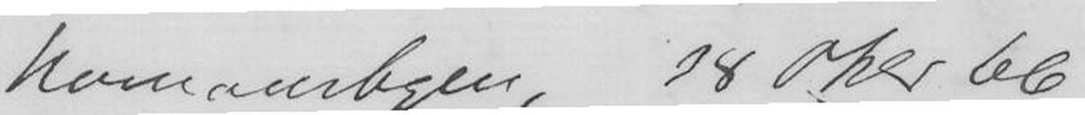Homansbyen, 18 Oktbr. 66
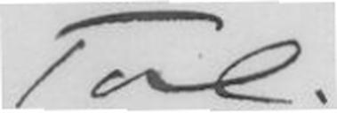Tal.
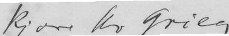Kjære Hr. Grieg
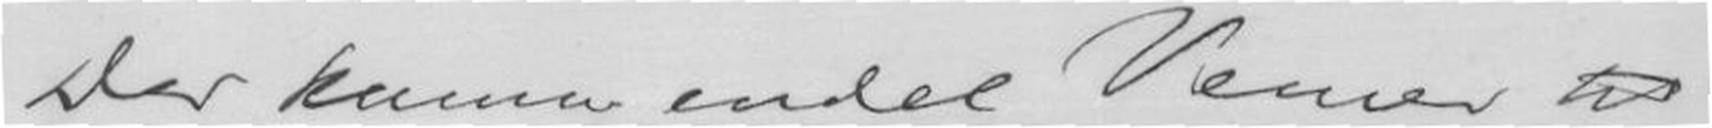Der kommer endel Venner til
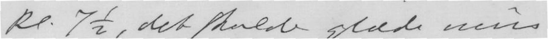kl. 7 1/2, det skulde glæde min
